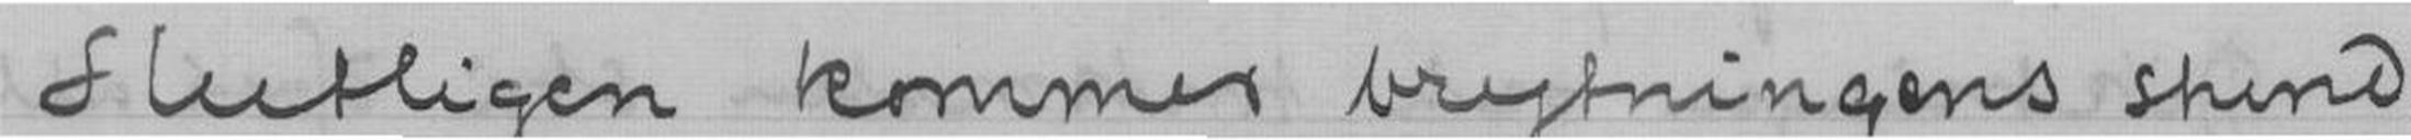Slutligen kommer brytningens stund,
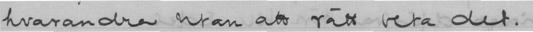hvarandra utan att rätt veta det.
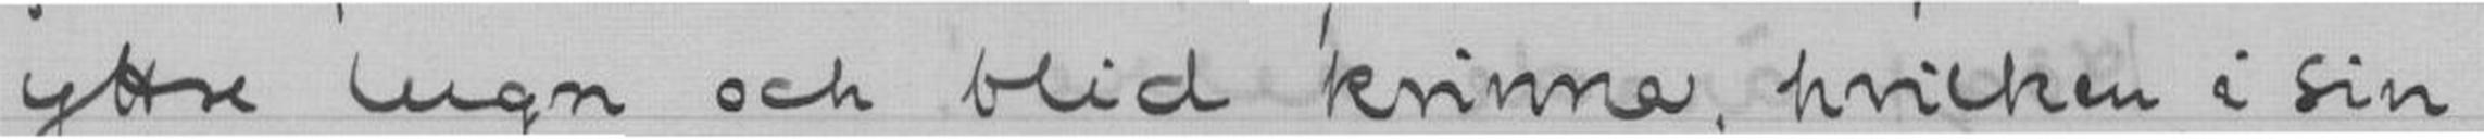yttre lugn och blid kvinna, hvilken i sin
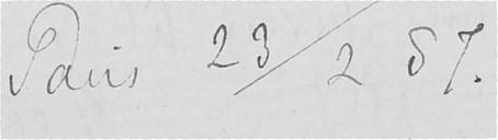Paris 23/2 87.
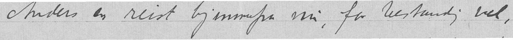Anders er reist hjemmefra nu, for bestandig vel,
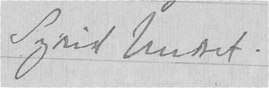Sigrid Undset.
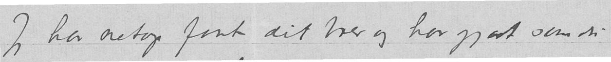Jeg har netop faat dit brev og har gjort som du
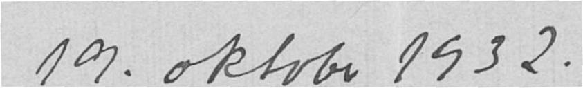19. oktober 1932.
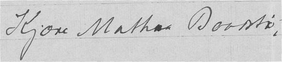Kjære Mathea Baadstø,
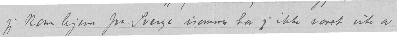jeg kom hjem fra Sverige isommer har jeg ikke været ute av
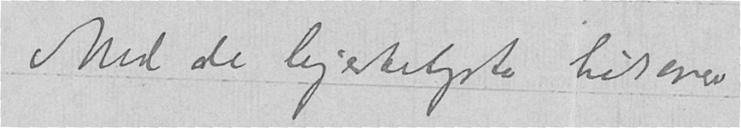Med de hjerteligste hilsener
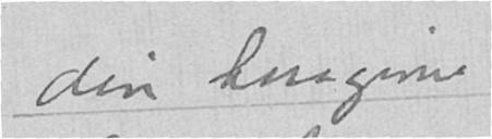din hengivne
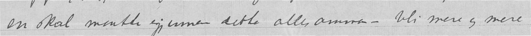en skal maatte igjennem dette allesammen – bli mere og mere
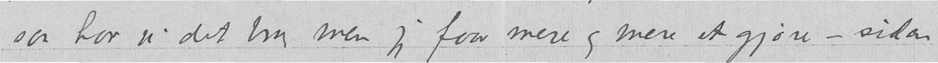saa har vi det bra, men jeg faar mere og mere at gjøre – siden
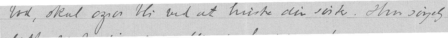bad, skal ogsaa bli ved at huske din søster. Hvor sørgelig
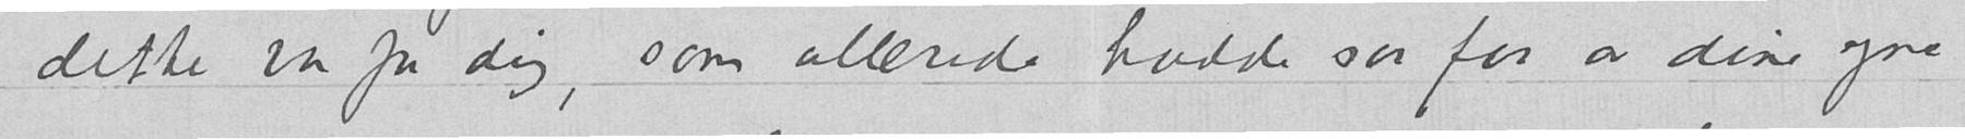dette var for dig, som allerede hadde saa faa av dine egne
